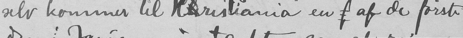selv kommer til Kristiania en af de første
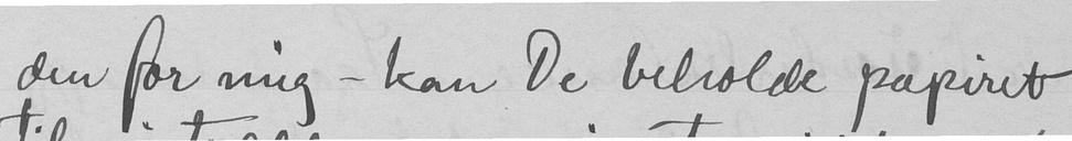den for mig - kan De beholde papiret
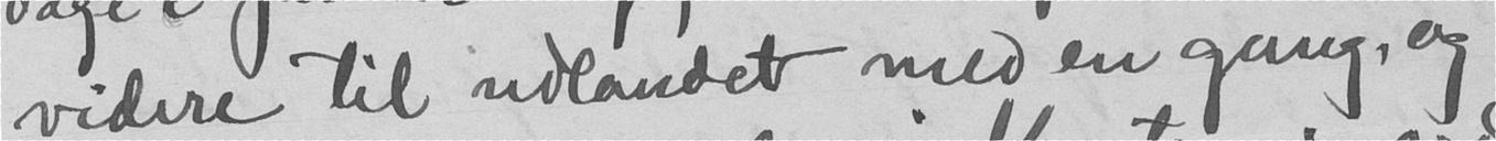videre til udlandet med en gang, og
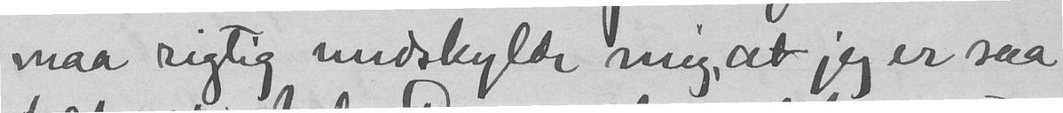maa rigtig undskylde mig, at jeg er saa
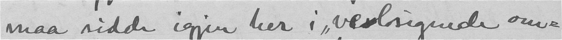maa sidde igjen her i "velsignede om-
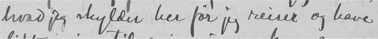hvad jeg skylder her før jeg reiser og have
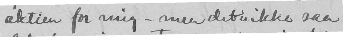aktien for mig - men det er ikke saa
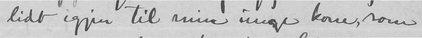lidt igjen til min min unge kone, som
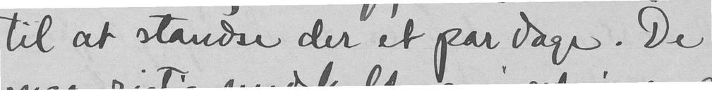til at standse der et par dage. De
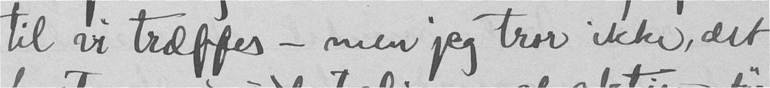til vi træffes, - men jeg tror ikke, det
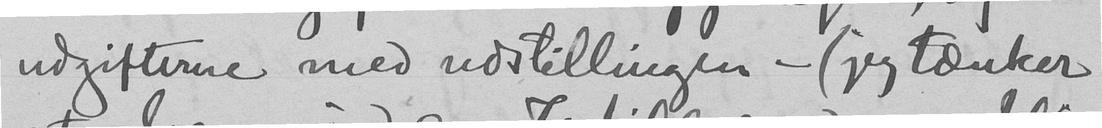udgifterne med udstillingen - (jeg tænker
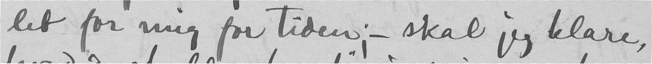let for mig for tiden; - skal jeg klare,
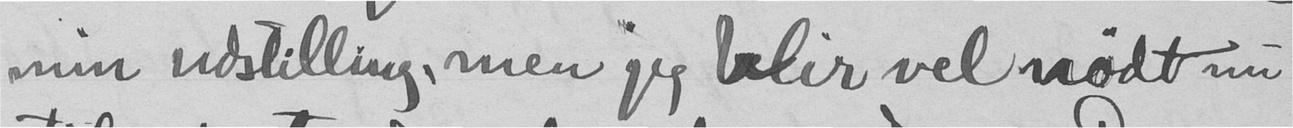min udstilling, men jeg blir vel nødt nu
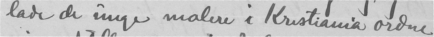lade de unge malere i Kristiania ordne
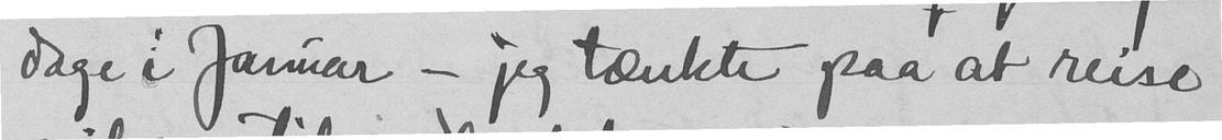dage i Januar - jeg tænkte paa at reise
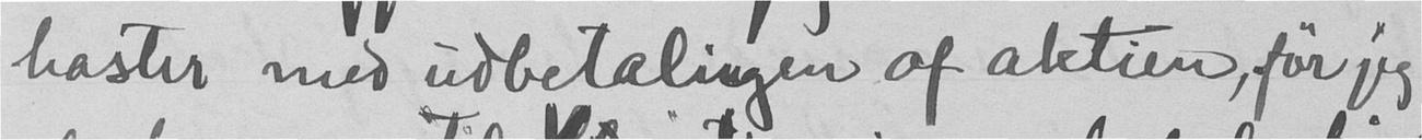haster med udbetalingen af aktien, før jeg
